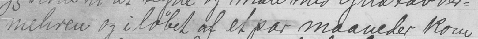mehren og i løbet af et par maaneder kom
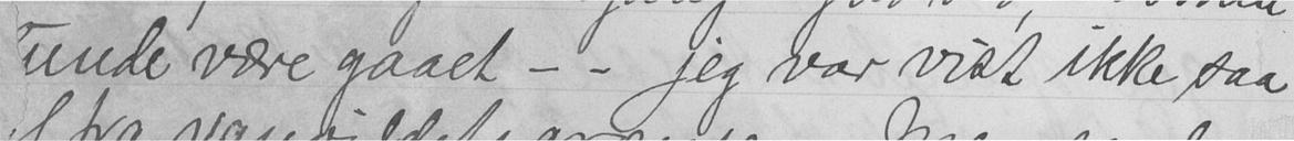unde være gaaet - - jeg var vist ikke saa
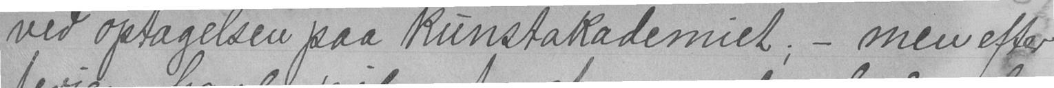ved optagelsen paa kunstakademiet; - men efter
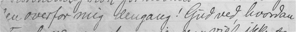en overfor mig dengang! Gud ved, hvordan
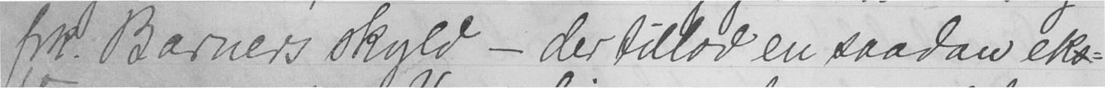frk. Barners skyld - der tillod en saadan eks-
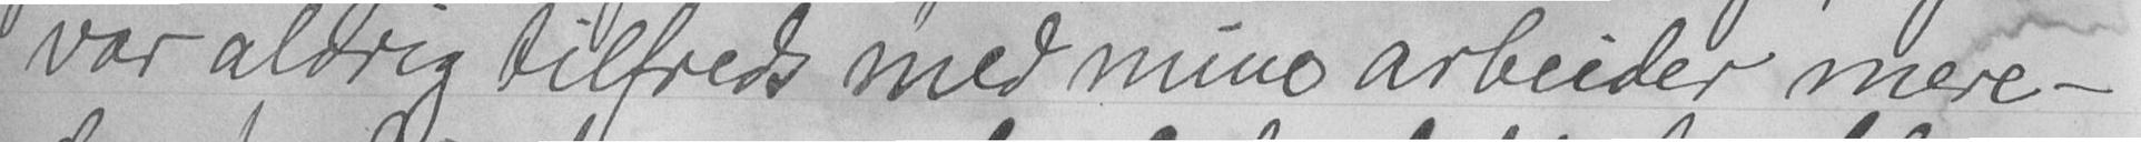var aldrig tilfreds med mine arbeider mere -
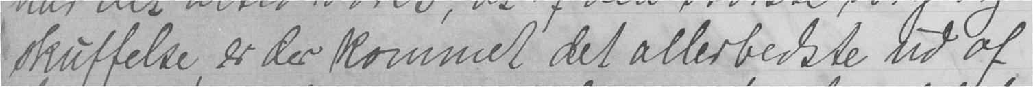skuffelse er der kommet det allerbedste ud af
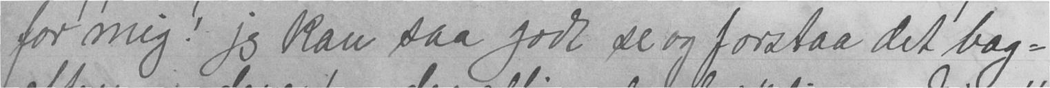for mig! jeg kan saa godt se og forstaa det bag-
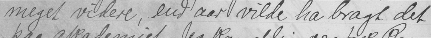meget videre, end aar vilde ha bragt det
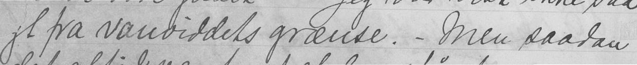gt fra vanviddets grænse. - Men saadan
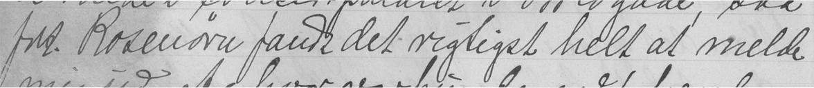frk. Rosenørn fandt det rigtigst helt at melde
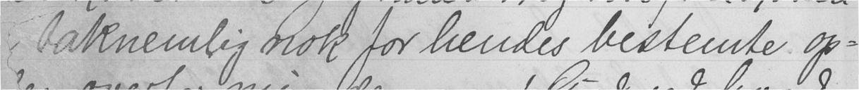qvist taknemlig nok for hendes bestemte op-
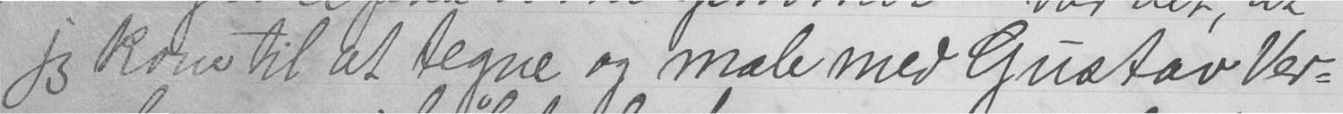jeg kom til at tegne og male med Gustav Ver-
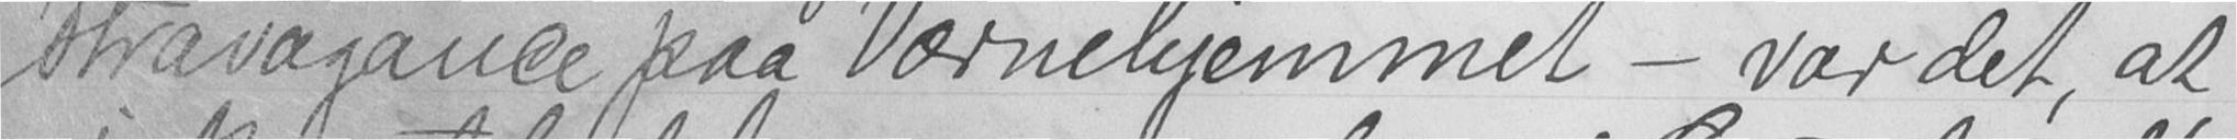travagance paa Værnehjemmet - var det, at
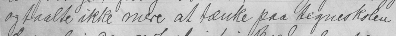og taalte ikke mere at tænke paa tegneskolen
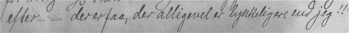efter - - der er faa, der alligevel er lykkeligere end jeg!!
